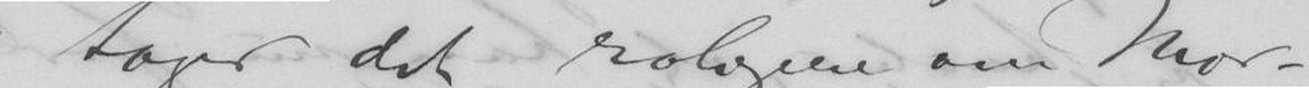jeg tager det roligere om Mor-
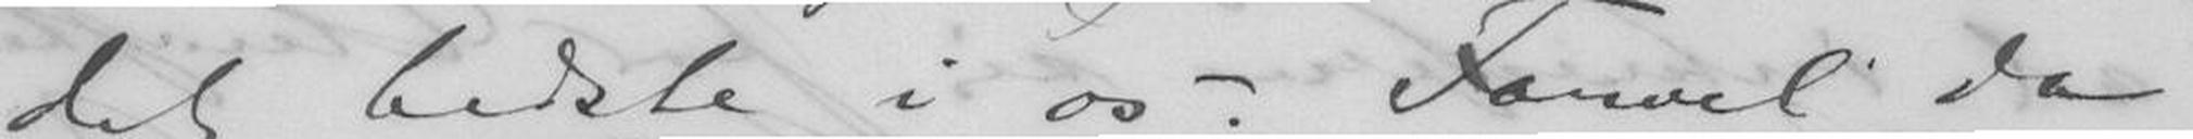det bedste i os. Farvel da,
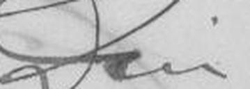Din
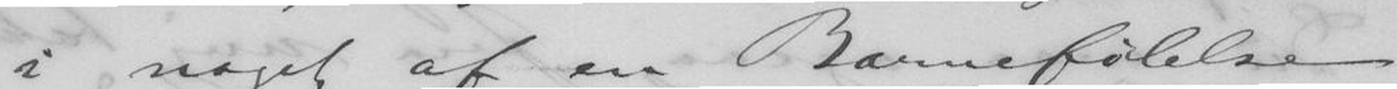i noget af en Barnefølelse,
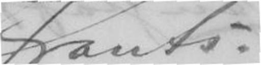Frants.
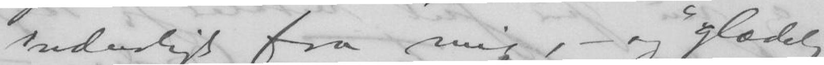inderligt fra mig, - og "glædelig
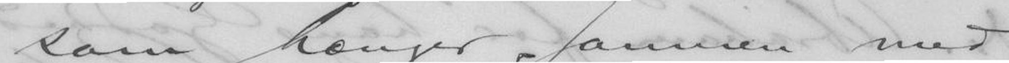som hænger sammen med
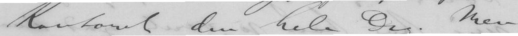Kontoret den hele Dag. Men
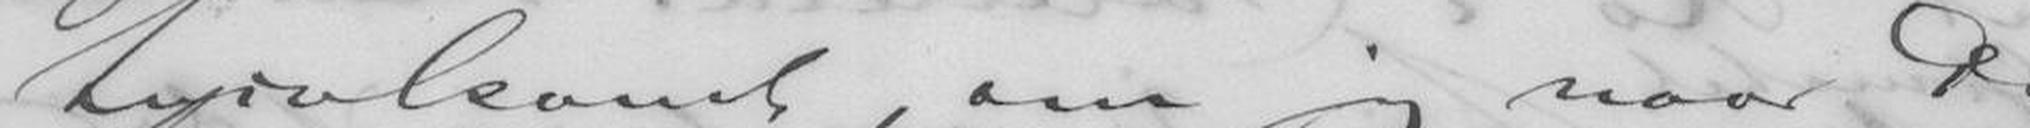tvivlsomt, om jeg naar Dig
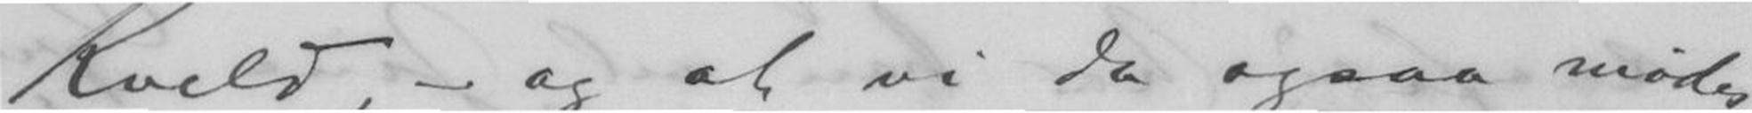Kveld, - og at vi da ogsaa mødes
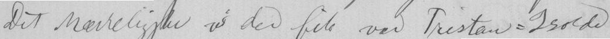Det Mærkeligste vi der fik var Tristan=Isolde
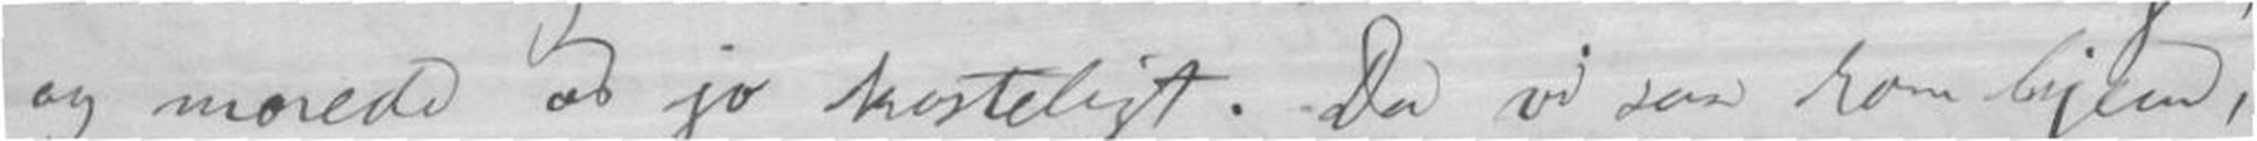og morede os jo kosteligt. Da vi saa kom hjem,
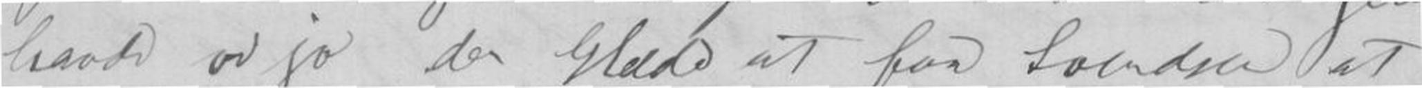havde vi jo den Glæde at faa Svendsen at
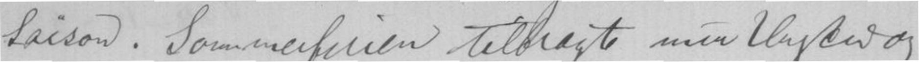Saison. Sommerferien tilbragte min Hustru og
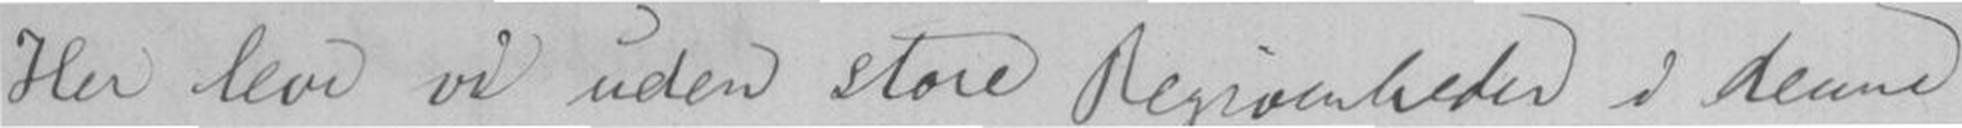Her lever vi uden store Begivenheder i denne
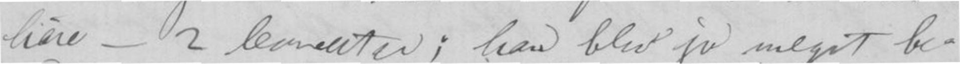høre - 2 Concerter; han blev jo meget be-
